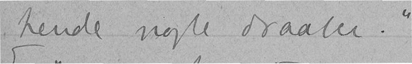hende nogle draaber."
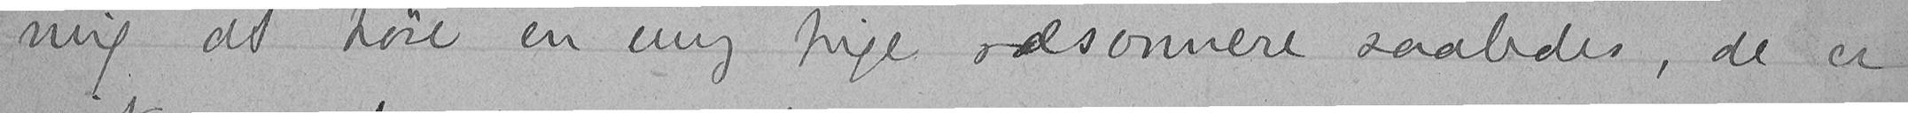mig at høre en ung pige ræsonnere saaledes, de er
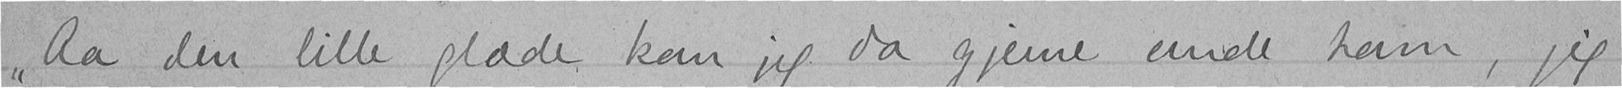"Aa den lille glæde kan jeg da gjerne unde ham, jeg
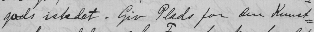gods istedet. Giv Plads for den Kunst-
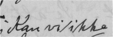Kan vi ikke
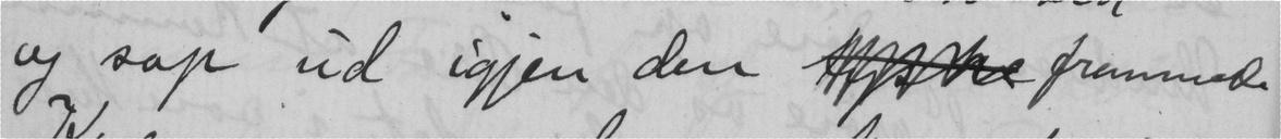og sop ud igjen den  fremmede
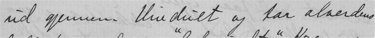ud gjennem Vinduet og tar alverdens
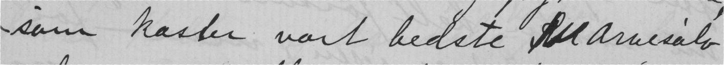som kaster vort bedste  arvesølv
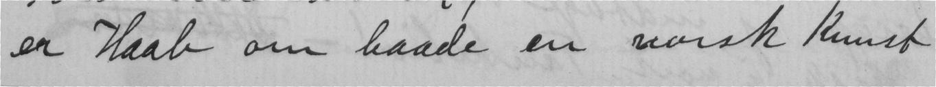er Haab om baade en norsk kunst
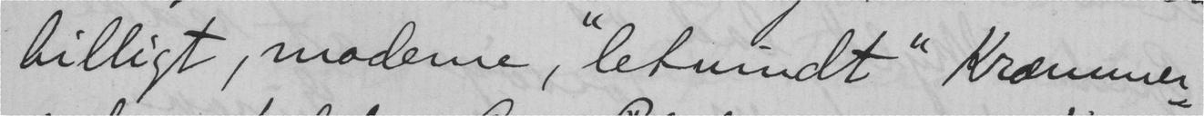billigt, moderne, "letvindt" Kræmmer-
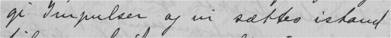gi Impulser og vi sættes istand
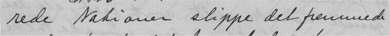rede Nationer slippe det fremmede
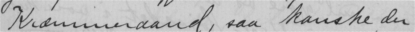Kræmmeraand, saa kanske der
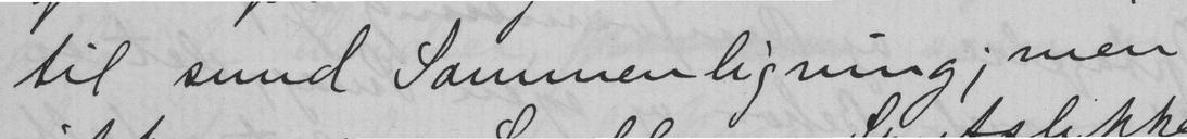til sund Sammenligning; men
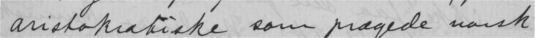aristokratiske som prægede norsk
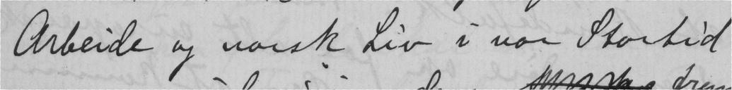Arbeide og norsk Liv i vor Stortid
